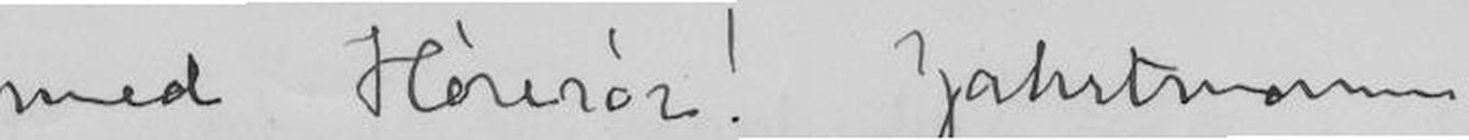med Hørerør! Jahrtmann
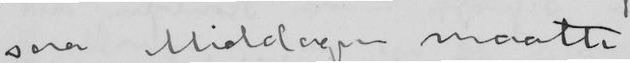saa Middagen maate
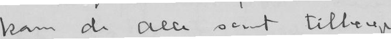kom de alle sent tilbage
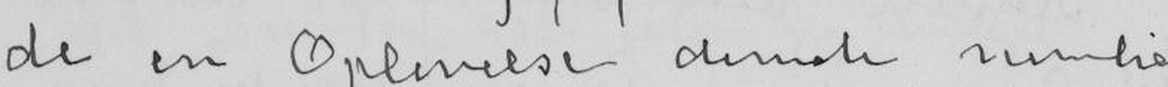de en Oplevelse derinde nemlig
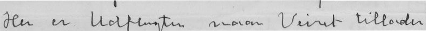Her er Udflugter naar Veiret tillader
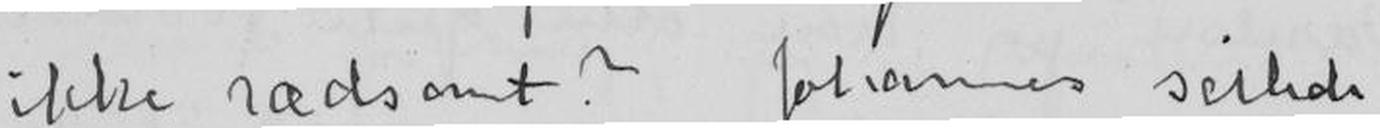ikke rædsomt? Johannes seilede
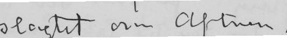slagtet om Aftnen.
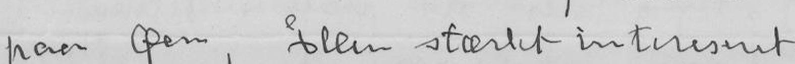paa Øen, Eller stærkt intereseret
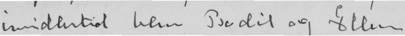imidlertid blev Bodil og Ellen
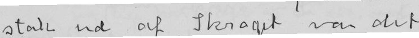stok ud af Skroget var det
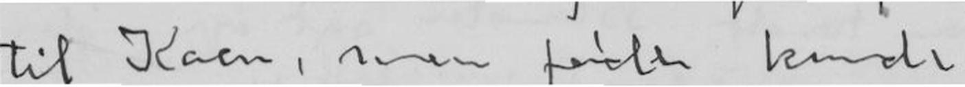til Koen, men føde kunde
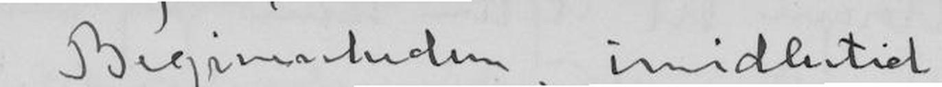i Begivenheden, imidlertid
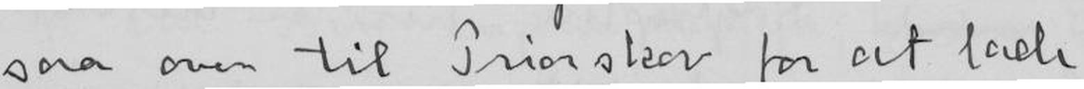saa over til Priorskor for at lade
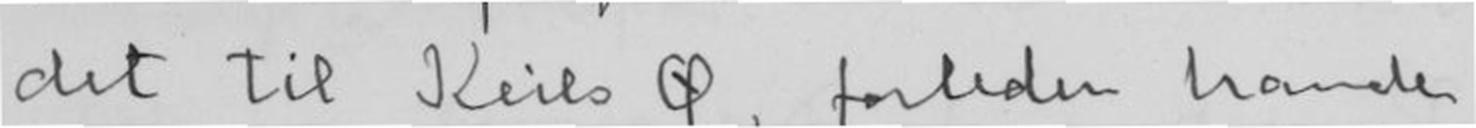det til Kiels Ø, forleden havde
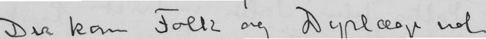Der kom Folk og Dyrlæge ud
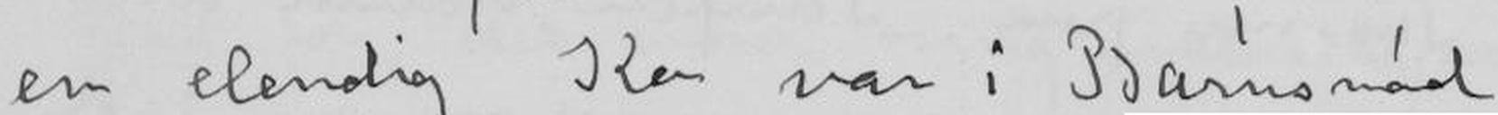er elendig Ko var i Barnsmod
